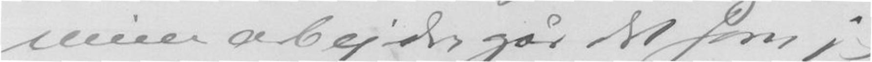mine arbejder går det som jeg
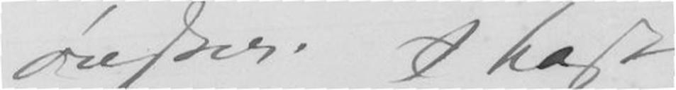ønsker. I hast
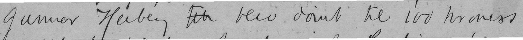Gunnar Heiberg fik blev dømt til 100 kroners
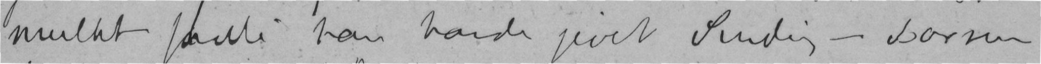mulkt fordi han havde givet Sinding-Larsen
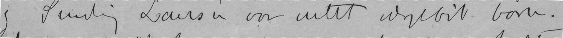og Sinding Larsen var intet værgeløst barn.
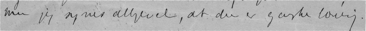men jeg synes alligevel, at den er ganske løelig.
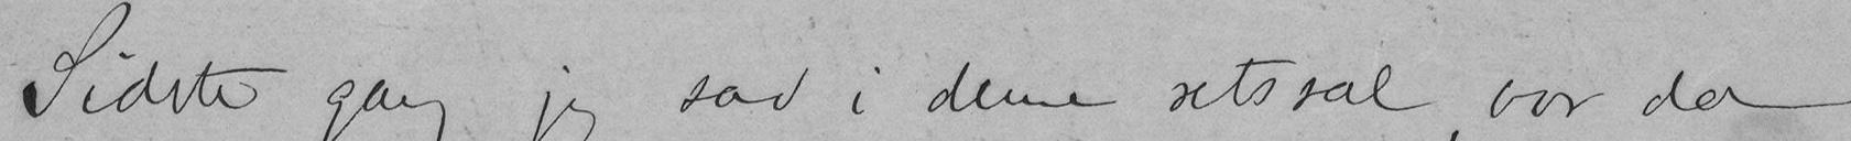Sidste gang jeg sad i dene retssal, var da
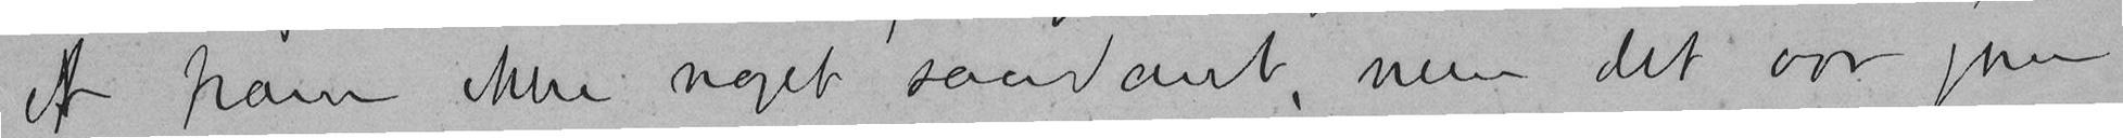et ham ikke noget saadant, men det var per
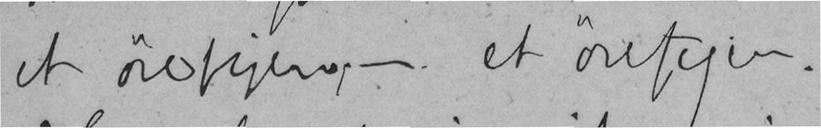et ørefigen - et ørefigen.
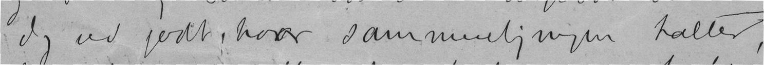Jeg ved godt, hvor sammeligningen halter,
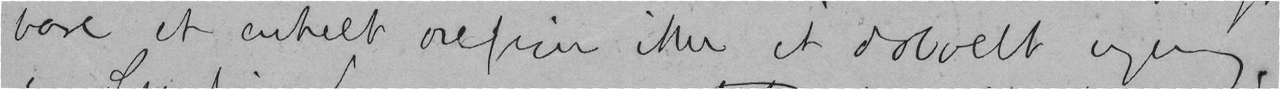bare et enkelt ørefigen ikke et dobbelt igen,
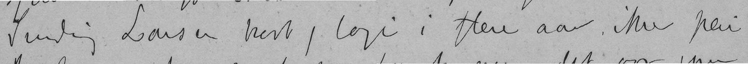Sinding Larsen kost, logi i flere aar, ikke plei
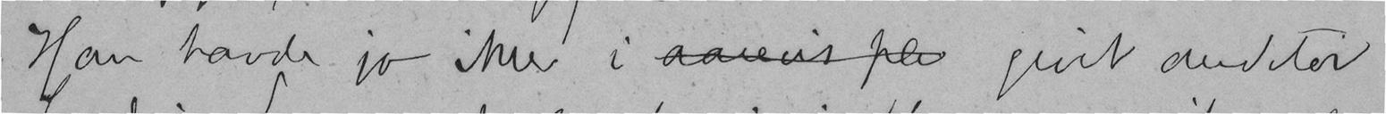Han havde jo ikke i aarevis ple givet audter
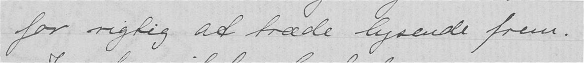for rigtig at træde lysende frem.
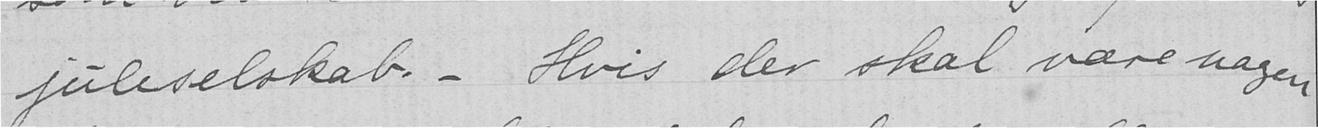juleselskab. - Hvis der skal være nogen
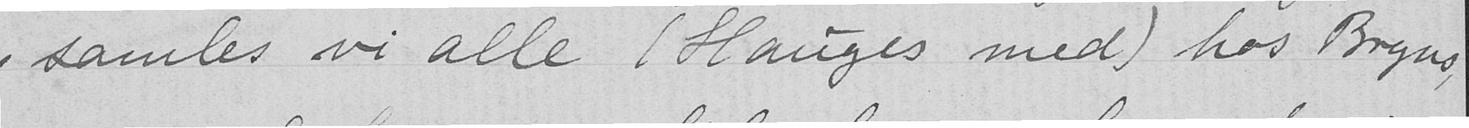samles vi alle (Hauges med) hos Bryns,
